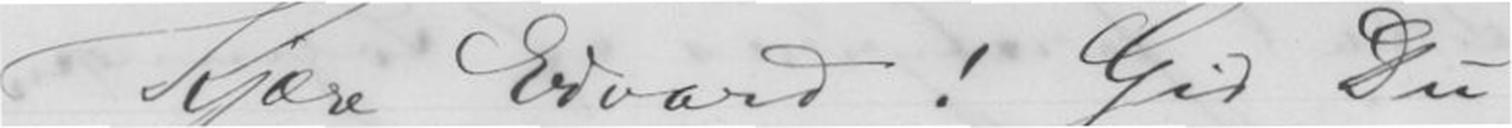Kjære Edvard! Gid Du
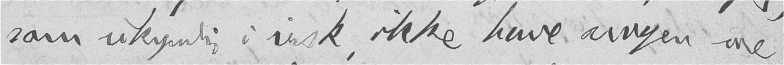som ukyndig i irsk, ikke have nogen me-
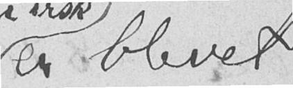er blevet
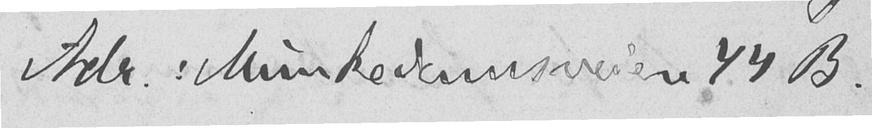Adr.: Munkedamsveien 44 B.
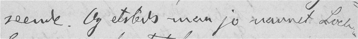seende. Og etsteds maa jo navnet Loch-
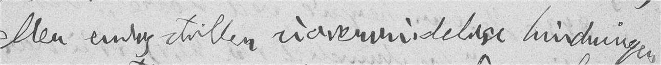eller endog stiller uovervindelige hindringer
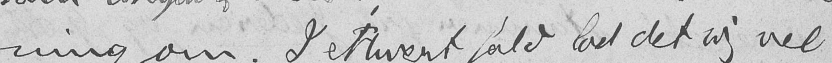ning om. I ethvert fald lod det sig vel
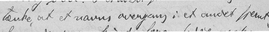tænke, at et navns overgang i et andet fjernt-
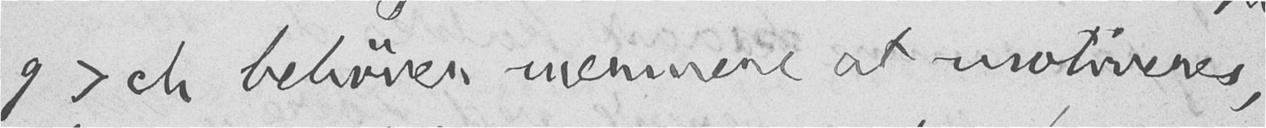g > ch behøver nærmere at motiveres,
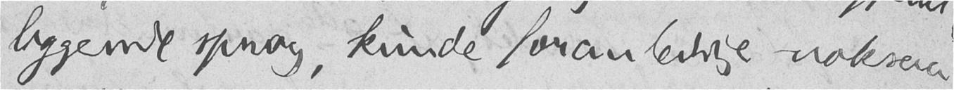liggende sprog, kunde foranledige noksaa
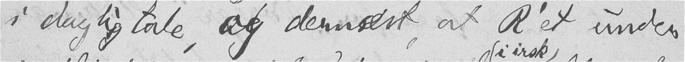i daglig tale, og dernæst, at R'et under
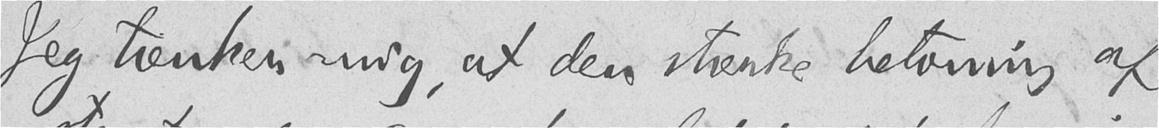Jeg tænker mig, at den stærke betoning af
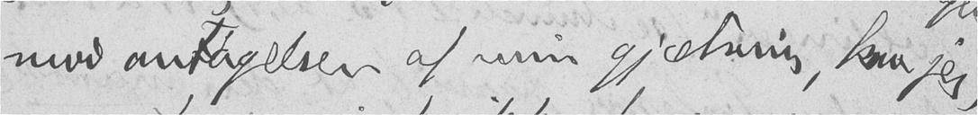mod antagelsen af min gjætning, kan jeg,
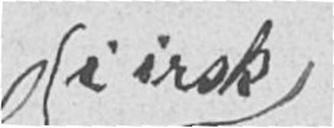i irsk
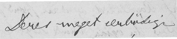Deres meget ærbødige
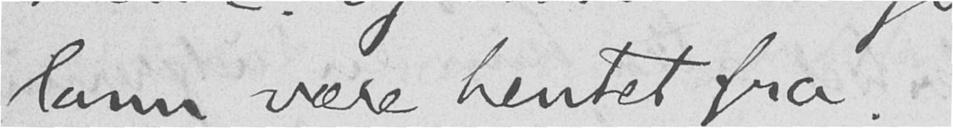lann være hentet fra.
